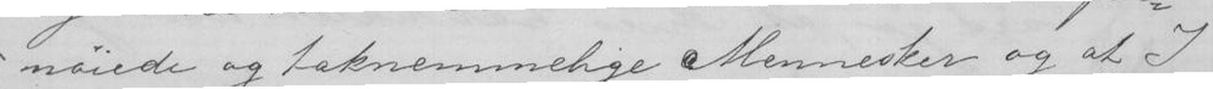nøiede og taknemmelige Mennesker og at I
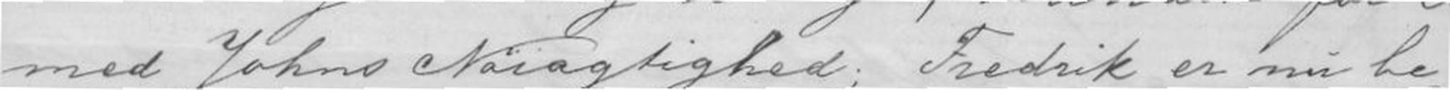med Johns Nøiagtighed; Fredrik er nu be-
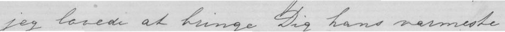jeg lovede at bringe Dig hans varmeste
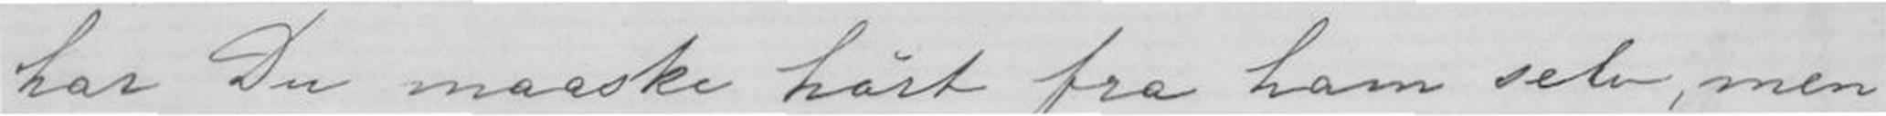har Du maaske hørt fra ham selv, men
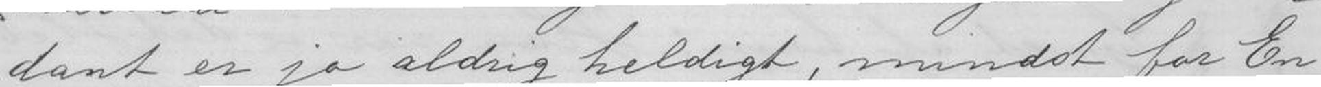dant er jo aldrig heldigt, mindst for En
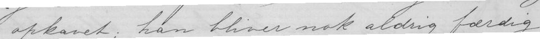opkavet; han bliver nok aldrig færdig
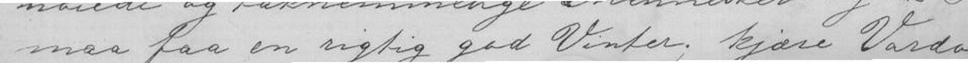maa faa en rigtig god Vinter; kjære Vardo
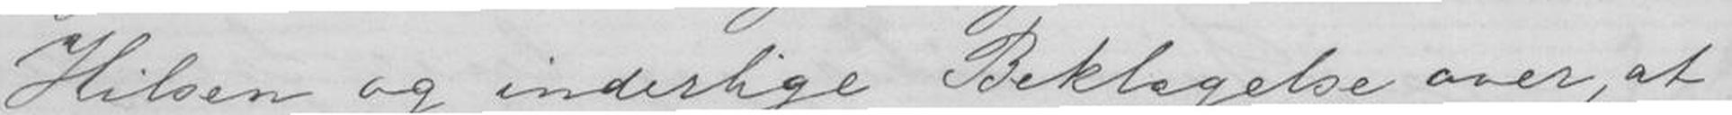Hilsen og inderlig Beklagelse over, at
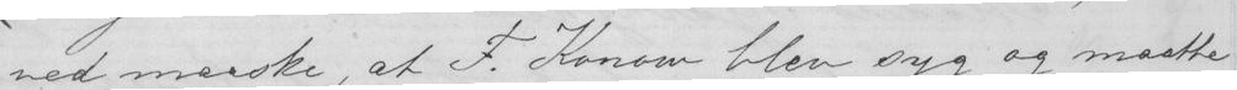ved maaske, at F. Konow blev syg og maatte
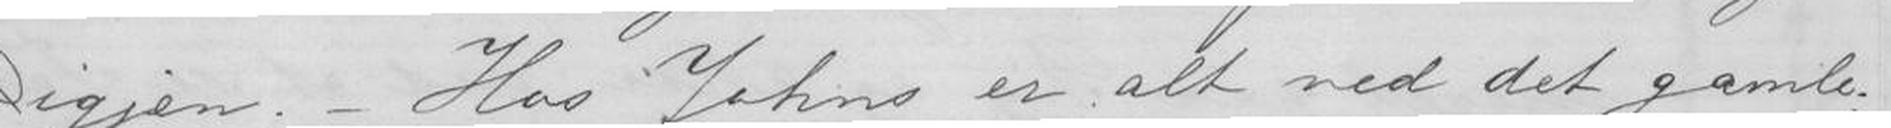igjen. - Hos Johns er alt ved det gamle;
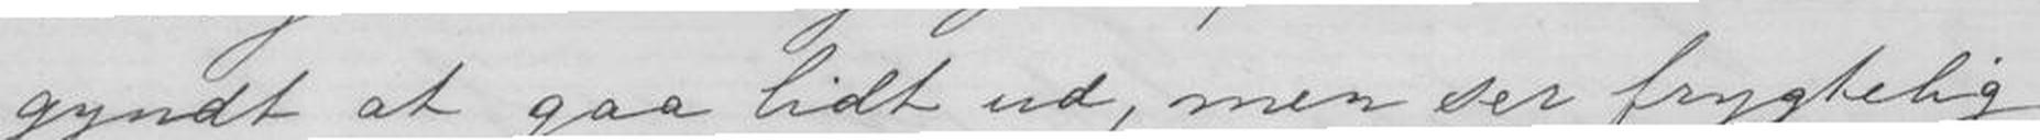gyndt at gaa lidt ud, men ser fryktelig
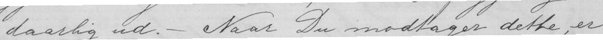daarlig ud. - Naar Du modtager dette, er
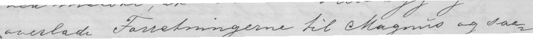overlade Forretningen til Magnus og saa-
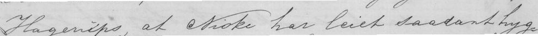Hagerups, at Niske har leiet saadant hyg-
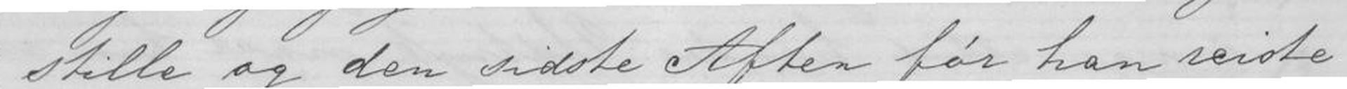stille og den sidste Aften før hen reiste
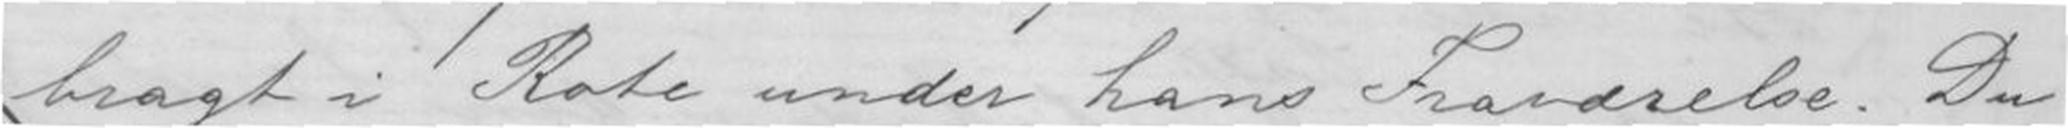bragt i Rote under hans Fraværelse; Du
